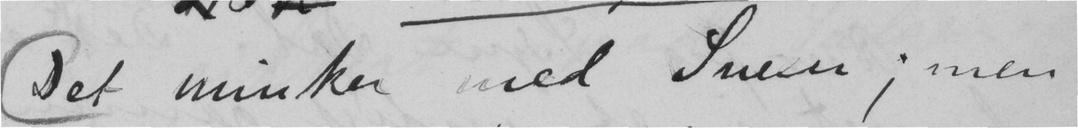Det minker med Sneen; men
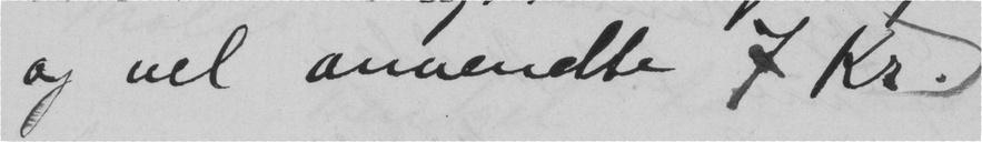og vel anvendte 7 Kr.
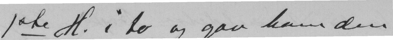1ste H. i to og gav ham den
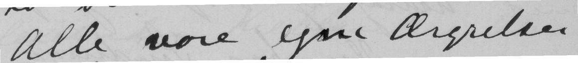Alle vore egne Ærgrelser
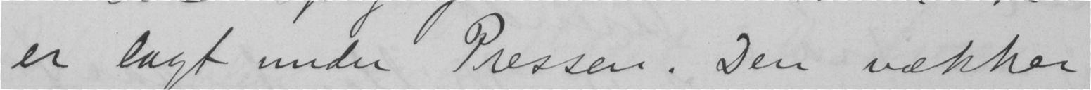er lagt under Pressen. Den vækker
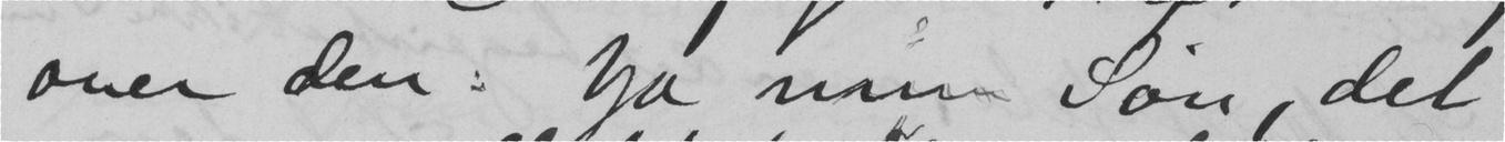over den: Ja min Søn, det
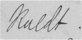kaldt.
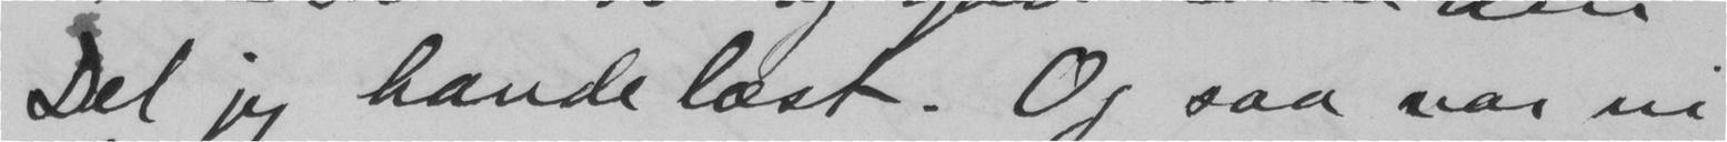Del jeg havde læst. Og saa var vi
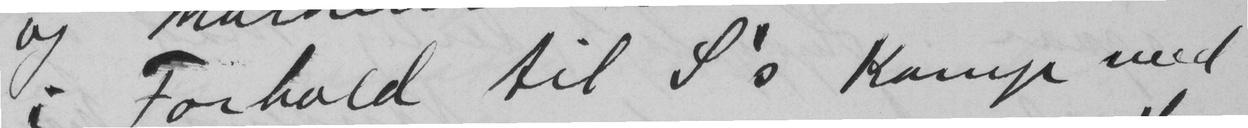i Forhold til S's Kamp med
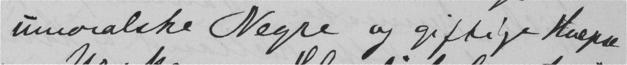umoralske Negre og giftige Hvepse
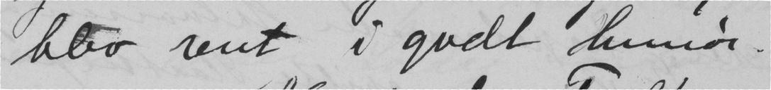blev rent i godt Humør.
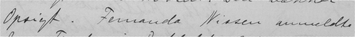Opsigt. Fernanda Nissen anmeldte
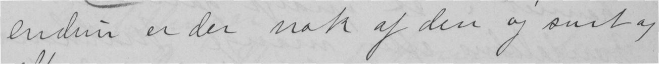endnu er der nok af den og surt og
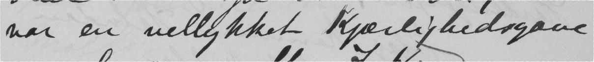var en vellykket Kjærlighedsgave
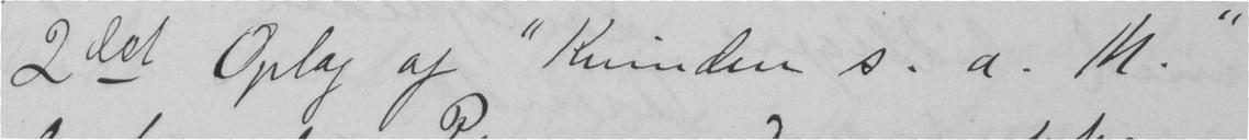2det Oplag af "Kvinden s. a. M."
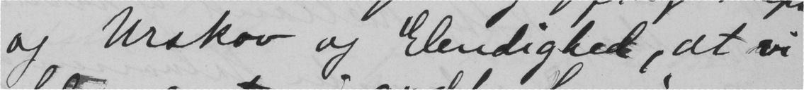og Urskov og Elendighed, at vi
